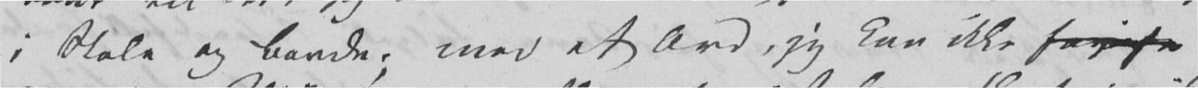i Stole og Borde; med et Ord, jeg kan ikke forvise
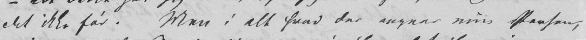det ikke før. Men i alt hvad der angaar min Person,
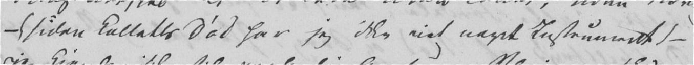– (siden Colletts Død har jeg ikke eiet noget Instrument) –
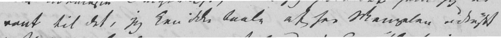vant til det, jeg kan ikke taale at see Mangelen udtrykt
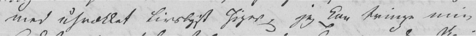med usvækket Livslyst higer, jeg kan tvinge min
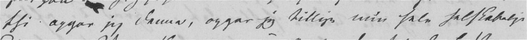thi opgav jeg denne, opgav jeg tillige min hele selskabelige
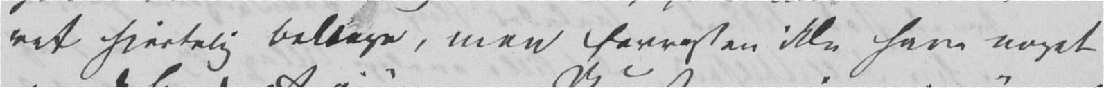ret hjertelig beklage, men forresten ikke have noget
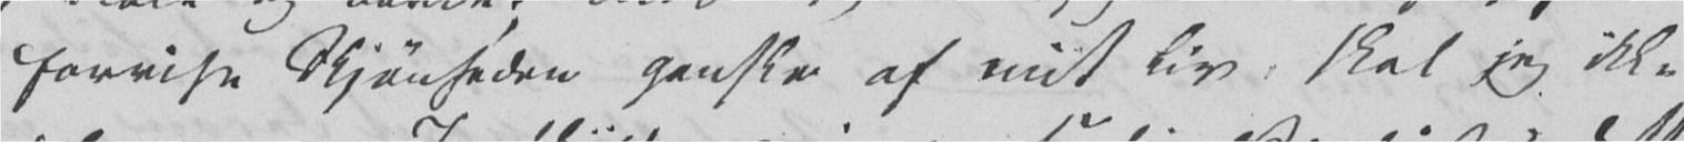forvise Skjønheden ganske af mit Liv, skal jeg ikke
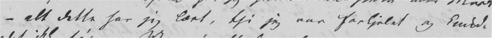– alt dette har jeg lært, thi jeg var forkjelet og kunde
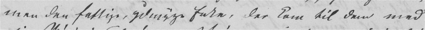men den fattige, ydmyge Enke, der kom til dem med
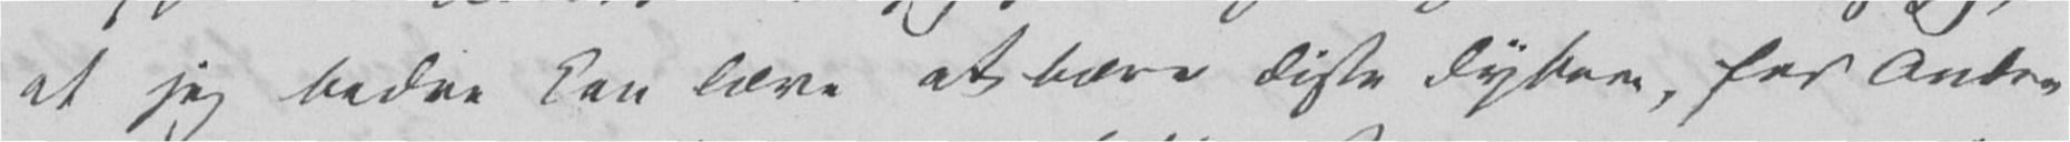at jeg bedre kan lære at bære disse dybere, for Andre
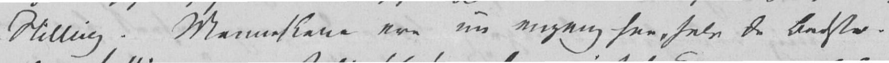Stilling. Menneskene ere nu engang saa, selv de Bedste.
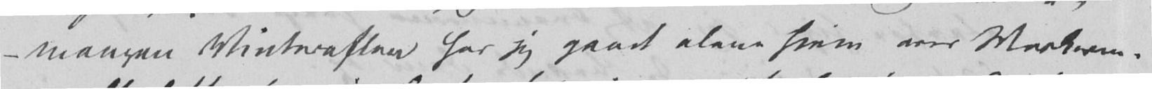– mangen Vinteraften har jeg gaaet alene hjem over Markerne.
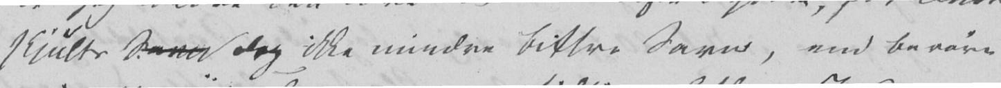skjulte Savn dog ikke mindre bittre Savn, end berøve
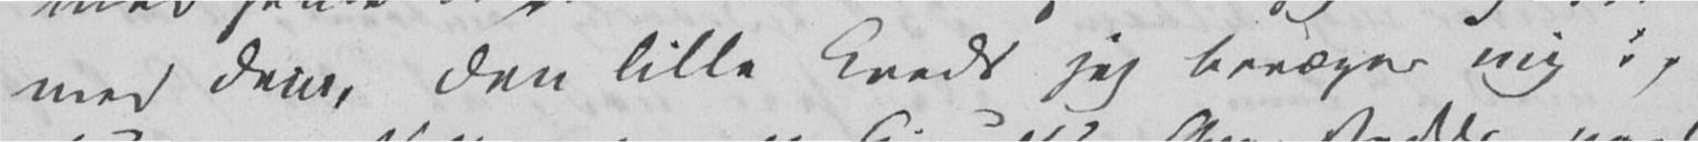med dem, den lille Kreds jeg bevæger mig i,
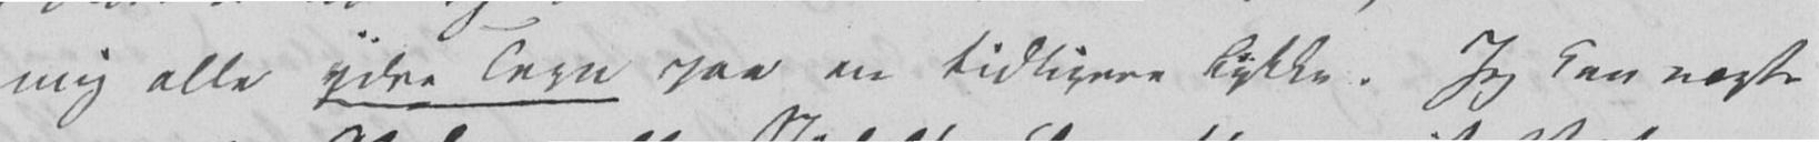mig alle ydre Tegn paa en tidligere Lykke. Jeg kan nægte
